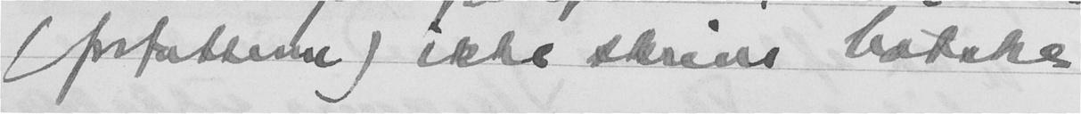(forfattere) ikke skrive hatske
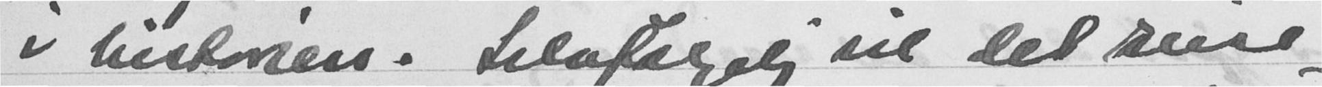i historien. Selvfølgelig vil det vise
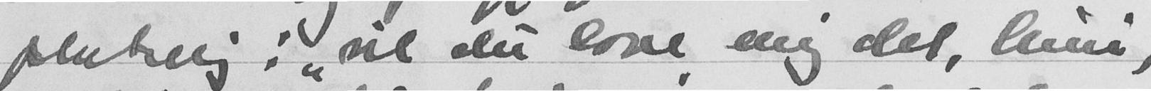plutselig: "vil du love mig det, Nini,
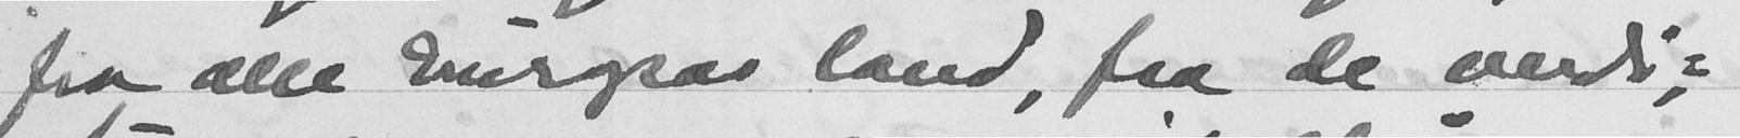fra alle Europas land, fra de verdi-
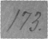173.
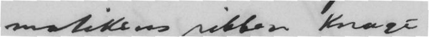matikens ribber knage -
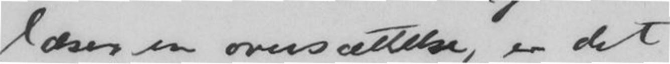læser en oversættelse, er det
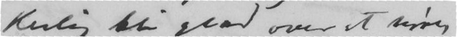kert bli glad over at høre
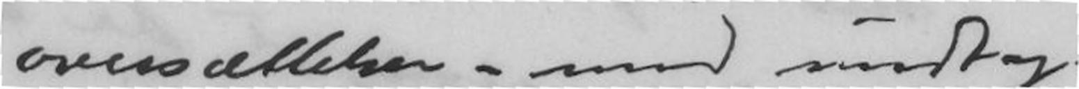oversættelse - mod undtag-
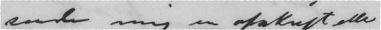sende mig en opskrift eller
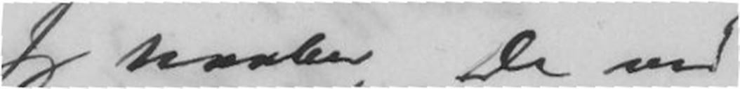Jeg haaber, De ved
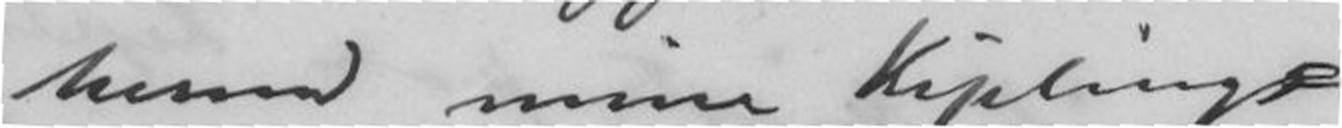hermed min Kiplings-
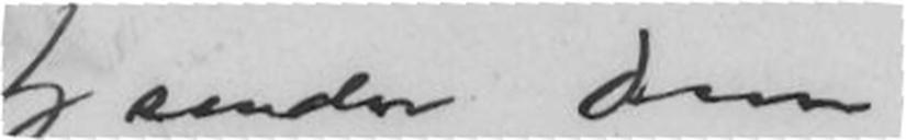Jeg sender dem
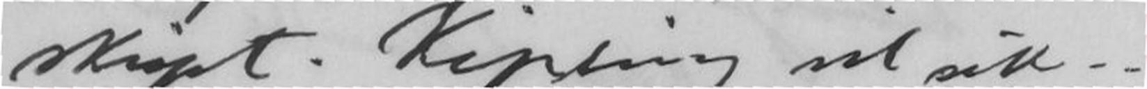skript. Kipling vil sik-
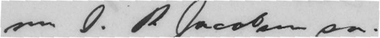som I. R. Jacobsen sa.
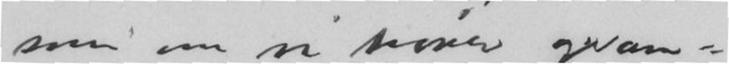som om vi hører gram-
